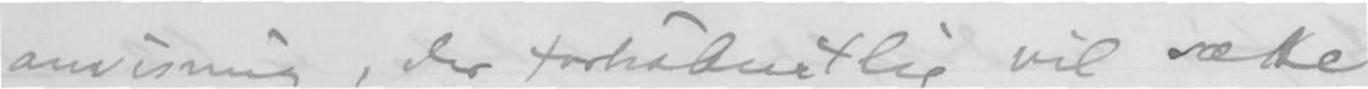anvisning, der forhåbentlig vil sætte
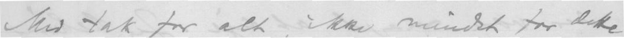Med tak for alt, ikke mindst for dette
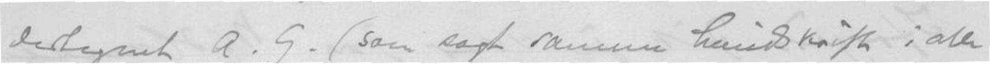dertegnet A.G. (som sagt samme håndskrift i alle
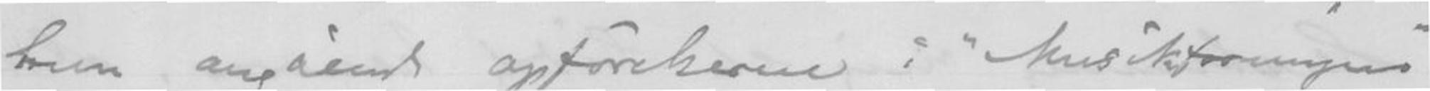hun angående opførelserne i "Musikforreningen"
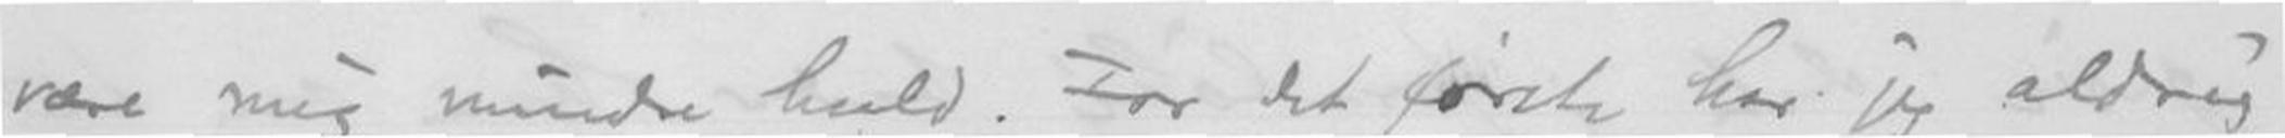være mig mindre huld. For det første har jeg aldrig
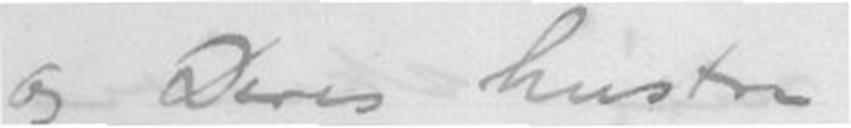og Deres hustru
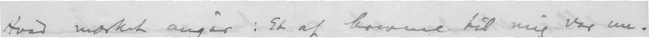Hvad mørket angår: Et af brevene til mig var un-
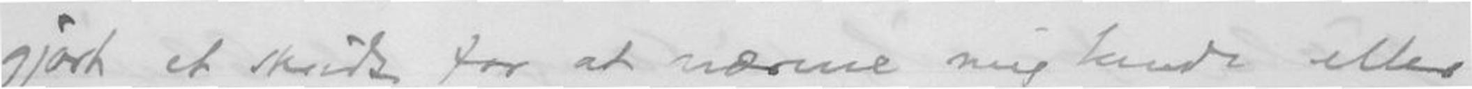gjort et skrids for at nærme mig hende eller
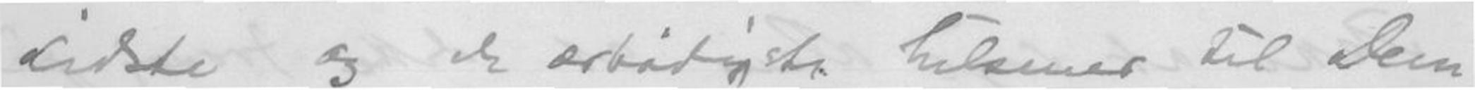sidste og de ærbødigste hilsener til Dem
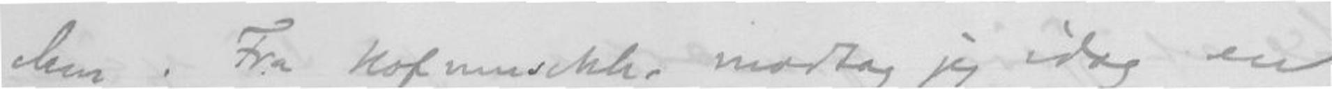ken. Fra hofmusikk modtog jeg idag en
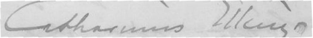Catharinus Elling
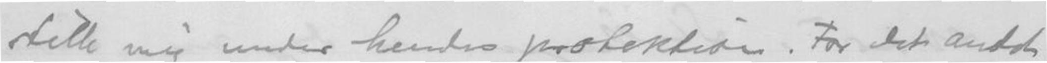stille mig under hendes protektion. For det andet
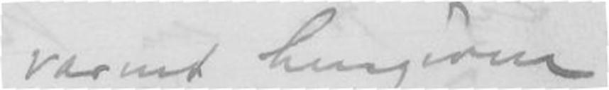varmt hengivne
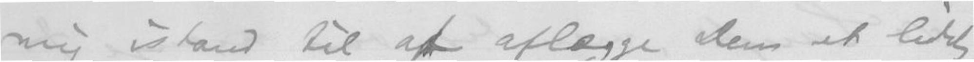mig istand til at aflægge dem et lidet
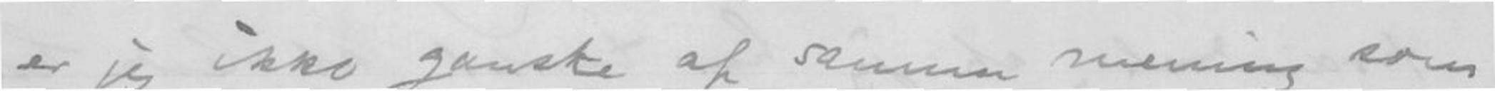er jeg ikke ganske af samme mening som
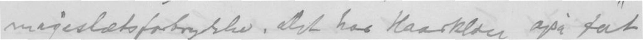majestætsforbrydelse. Det har Haarklou også fåt
